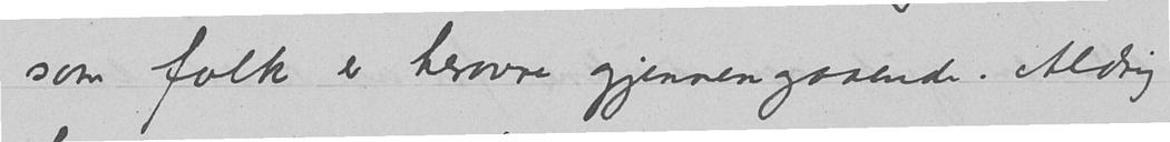som folk er herovre gjennemgaaende. Aldrig
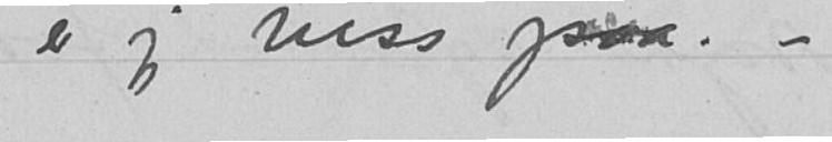er jeg viss paa. –
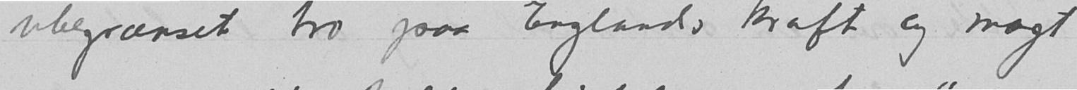ubegrænset tro paa Englands kraft og magt
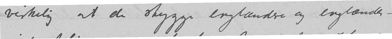virkelig at de stygge englændere og englænder-
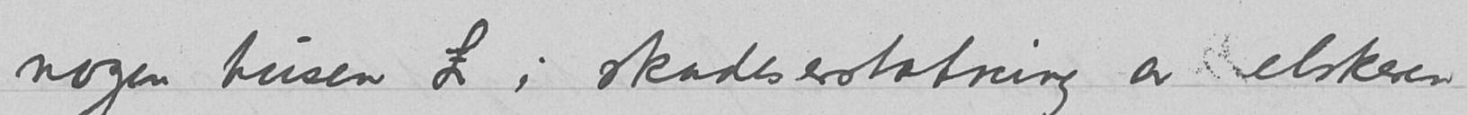nogen tusen £  i skadeserstatning av elskeren
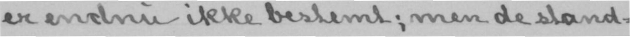er endnu ikke bestemt; men de stand-
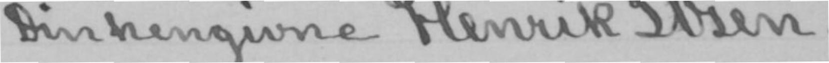Din hengivne Henrik Ibsen.
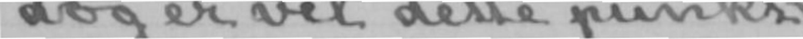dog er vel dette punkt
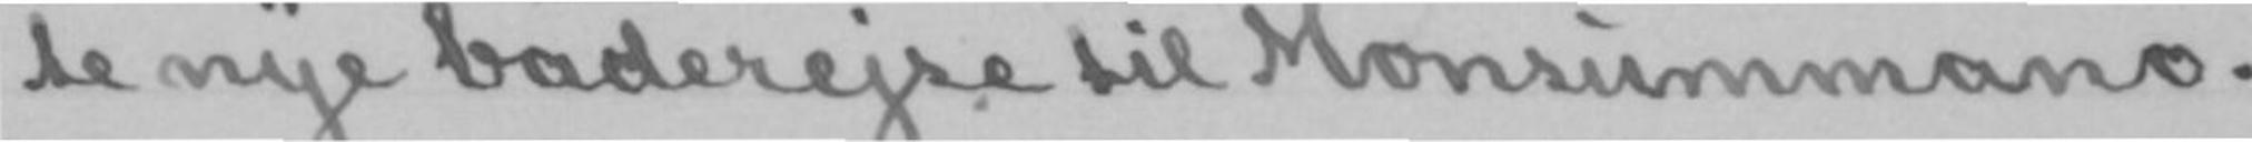te nye baderejse til Monsummano.
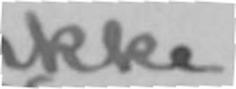ikke
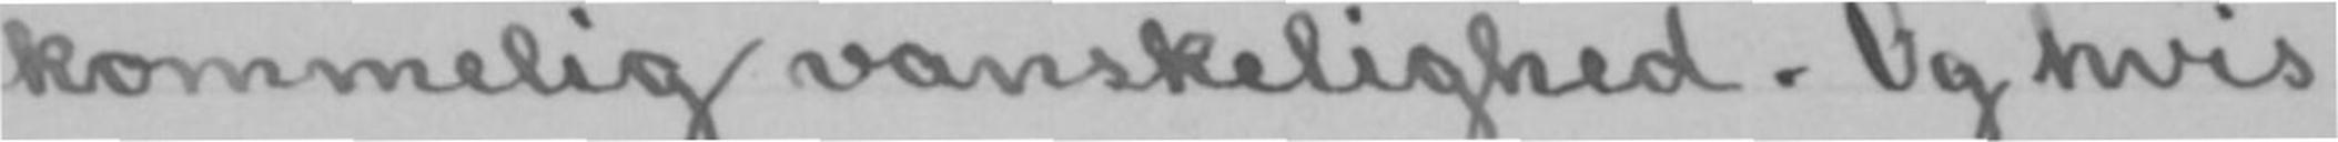kommelig vanskelighed. Og hvis
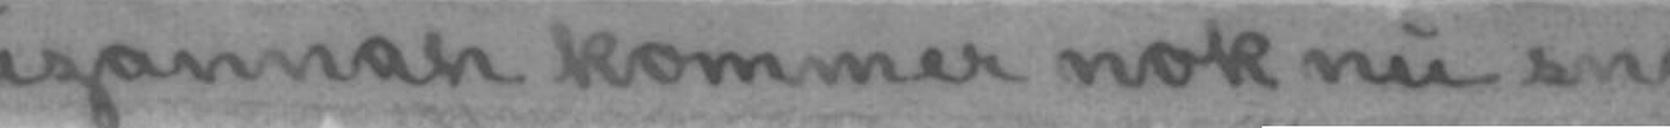Suzannah kommer nok nu snart
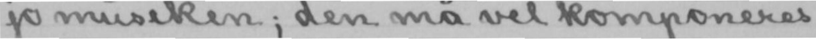jo musiken; den må vel komponeres;
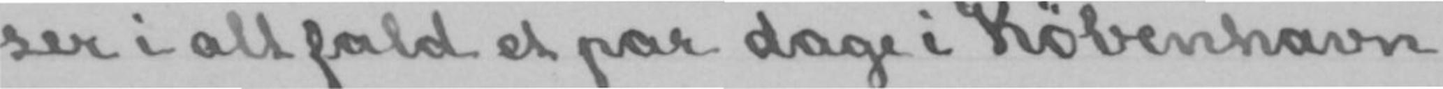ser i alt fald et par dage i København
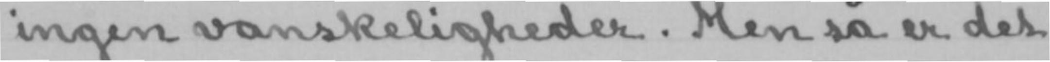ingen vanskeligheder. Men så er det
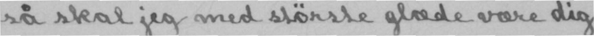så skal jeg med største glæde være dig
